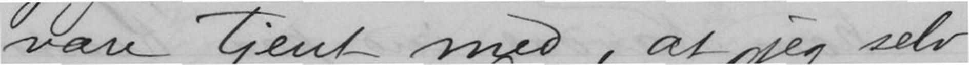være tjent med, at jeg selv
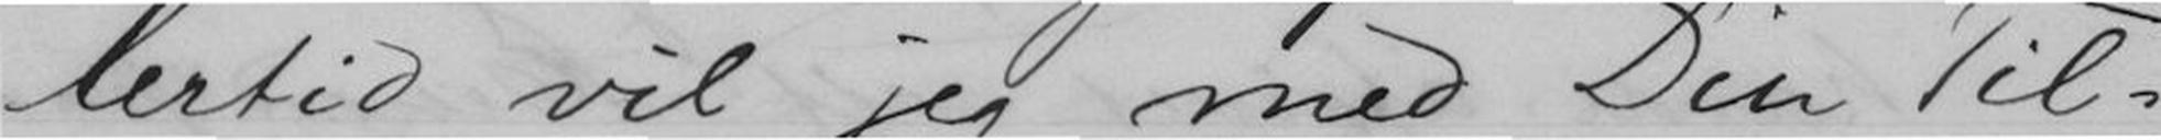lertid vil jeg med Din Til-
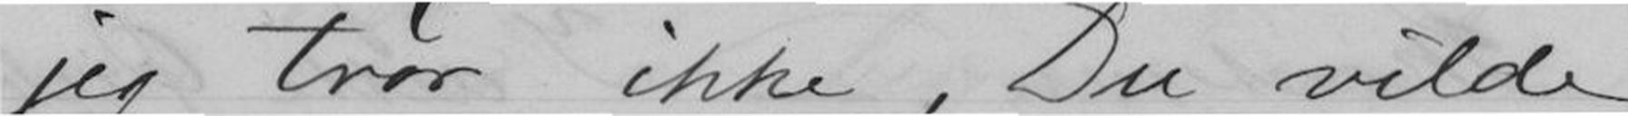jeg tror ikke, Du vilde
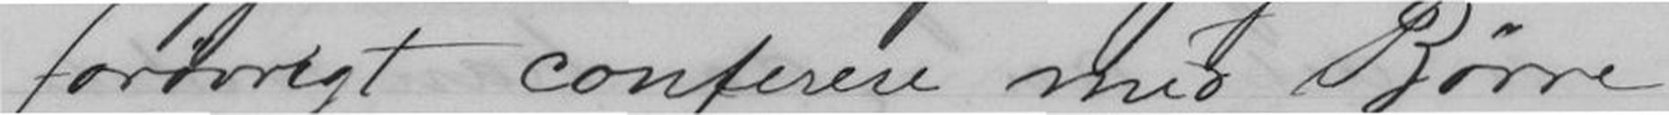forøvrigt conferer med Børre
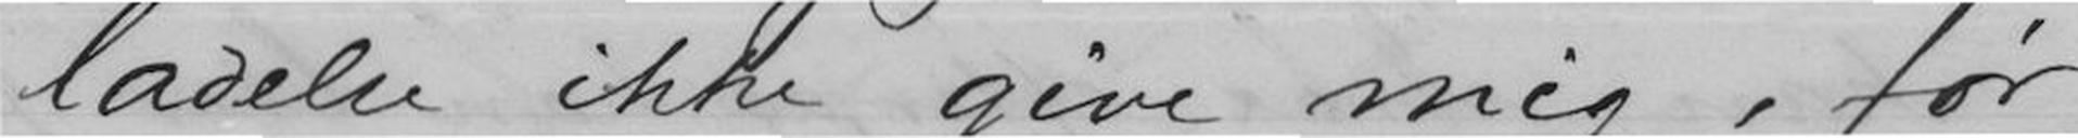ladelse ikke give mig, før
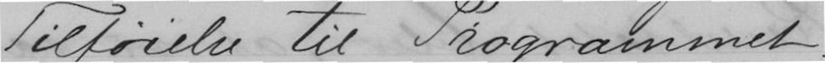Tilføielse til Programmet.
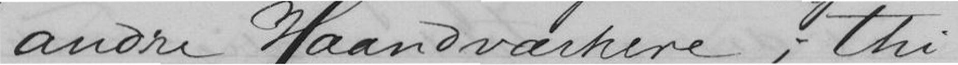andre Haandværkere; thi
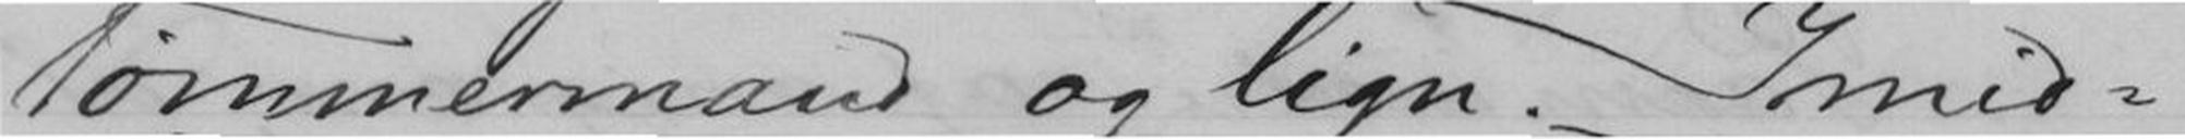Tømmermand og lign. Imid-
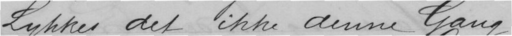Lykkes det ikke denne Gang
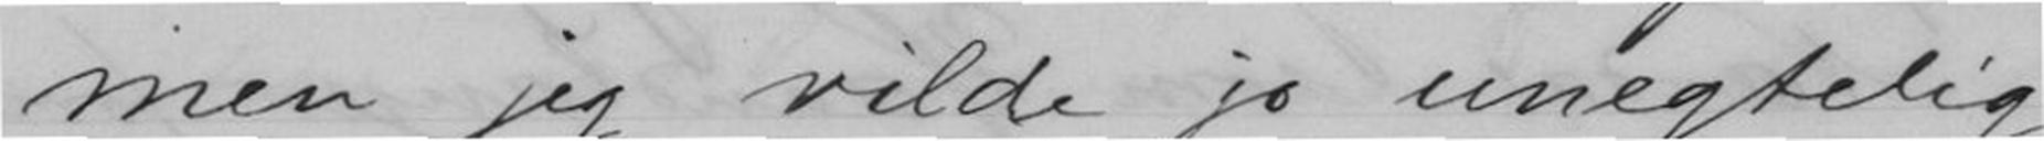men jeg vilde jo unegtelig
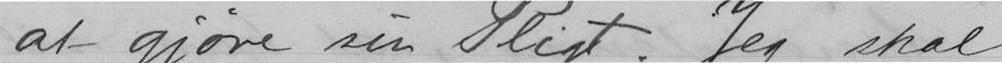at gjøre sin Pligt. Jeg skal
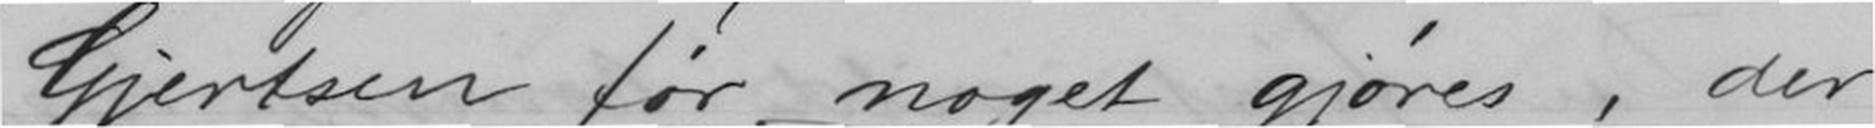Gjertsen før noget gjøres, der
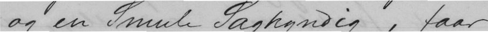og en Smule Sagkyndig, faar
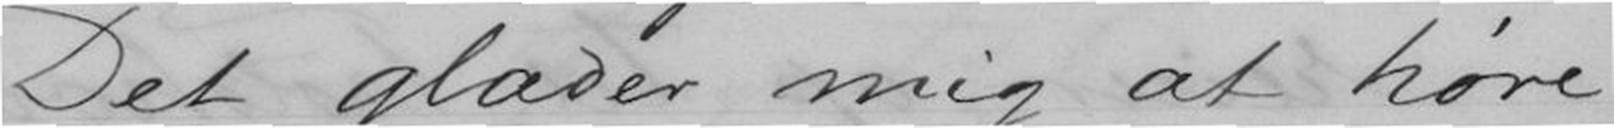Det glæder mig at høre,
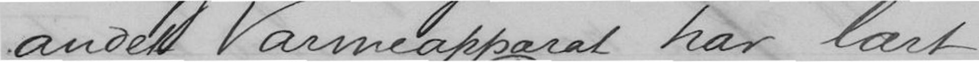andet Varmeapparat har lært
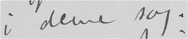i denne sag.
